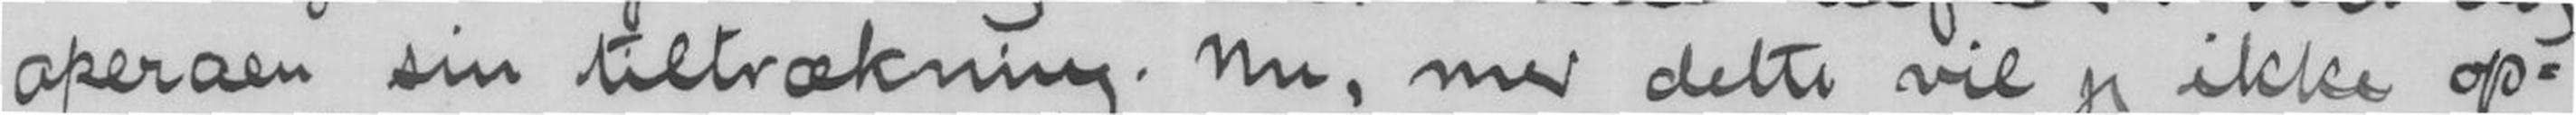operaen sin tiltrækning. Nu, med dette vil jeg ikke op-
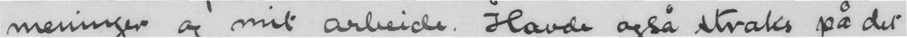meninger og mit arbeide. Havde også straks på det
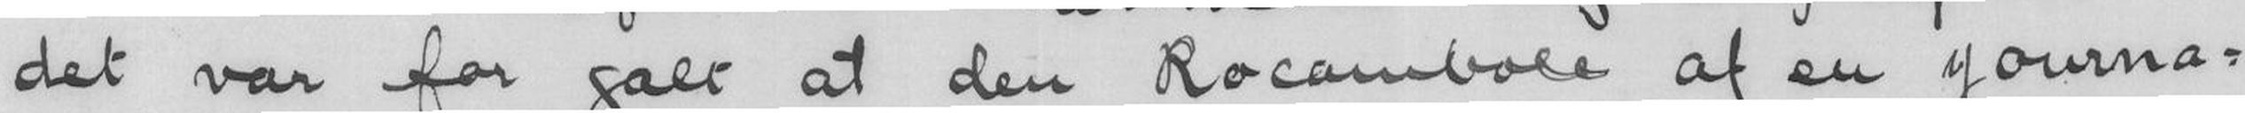det var for galt at den Rocambole af en journa-
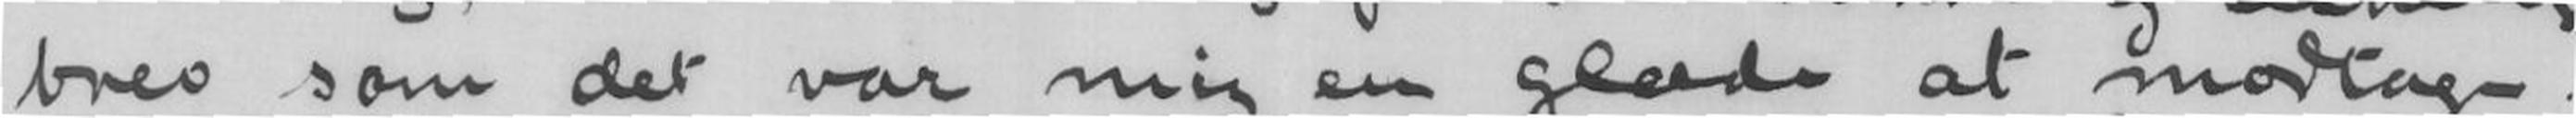brev som det var mig en glæde at modtage.
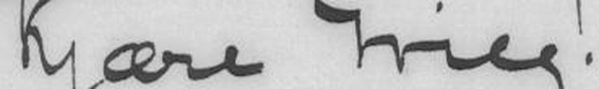Kjære Grieg!
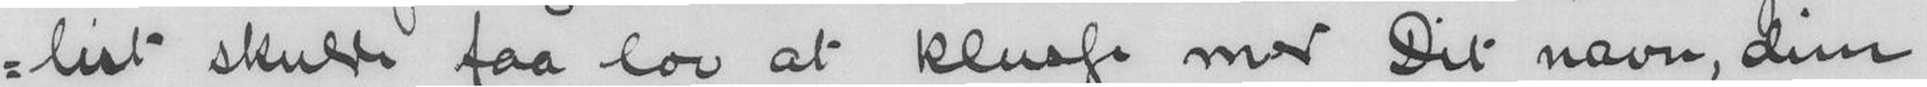list skulde faa lov at klusse med Dit navn, dine
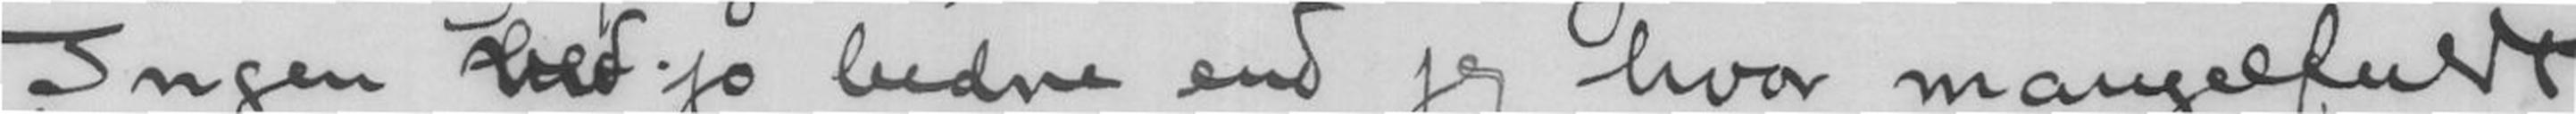Ingen ved jo bedre end jeg hvor mangelfuldt
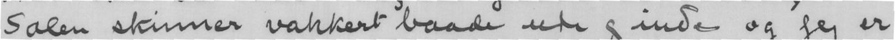Solen skinner vakkert baade ude og inde og jeg er
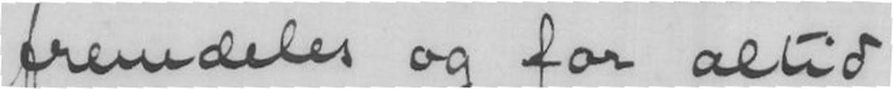fremdeles og for altid
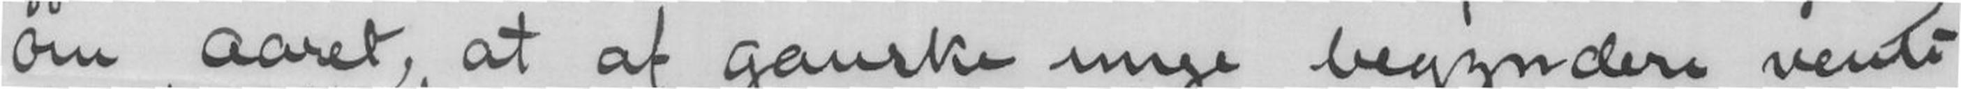om aaret, at af ganske nye begyndere vente
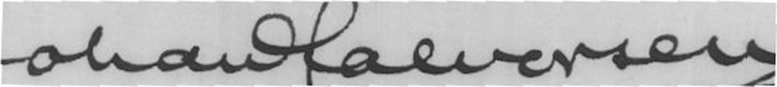Johan Halvorsen
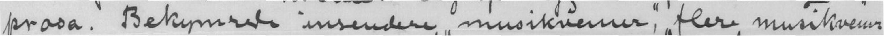prosa. Bekymrede insendere, musikvenner, flere musikvenner
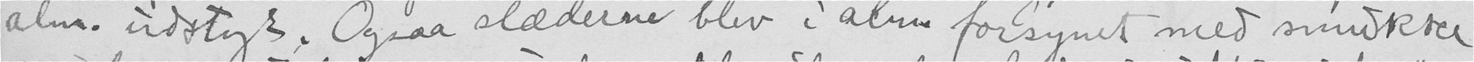alm. udstyr. Ogsaa slæderne blev i alm. forsynet med smukke
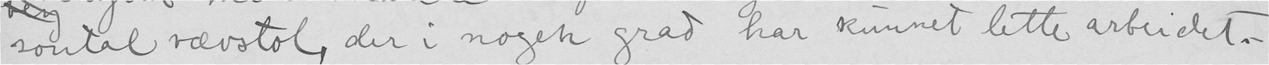sontal vævstol der i nogen grad har kunnet lette arbeidet.
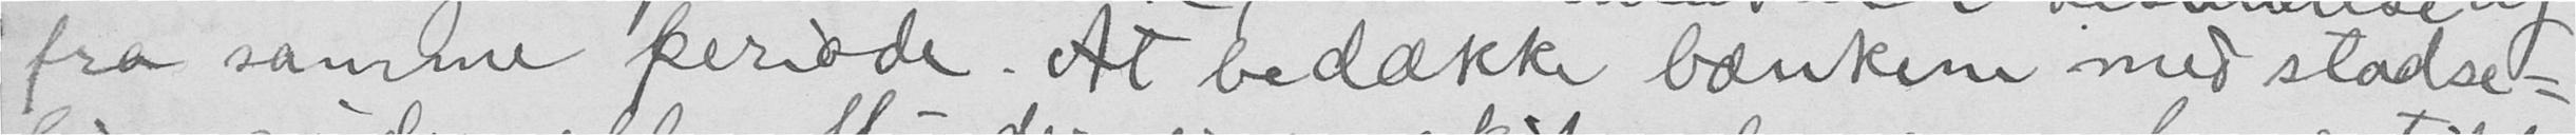fra samme periode. At bedække bænkene med stadse-
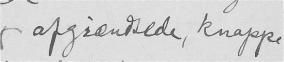afgrændsede, knappe
